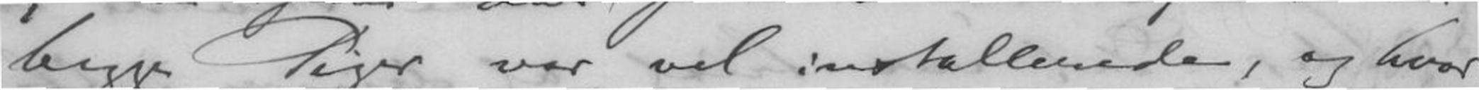begge Piger var vel installerede, og hvor
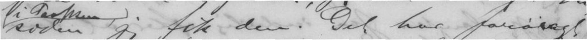i Paasken siden jeg fik den. Det har forøvrigt
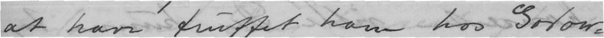at have truffet ham hos Godow-
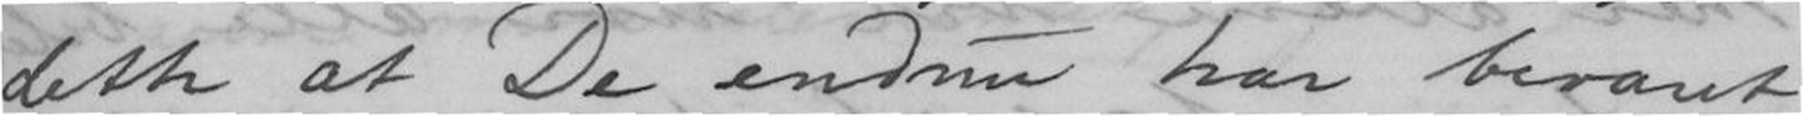dette at De endnu har bevaret
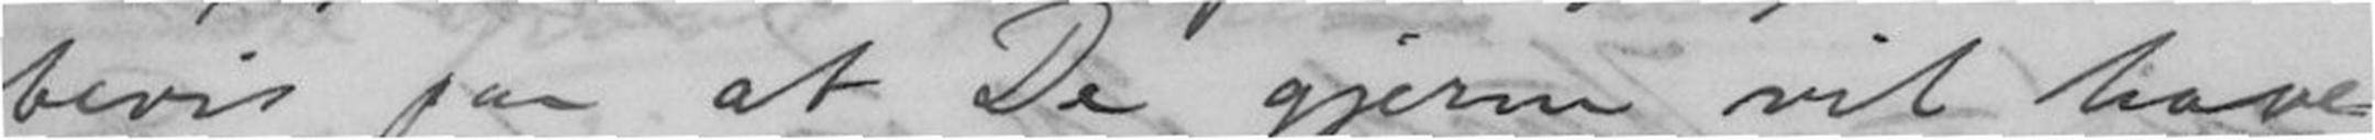bevis paa at De gjerne vil have
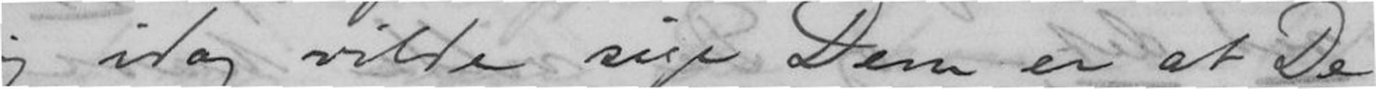jeg idag vilde sige Dem er at De
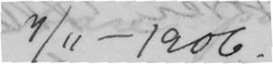7/11-1906
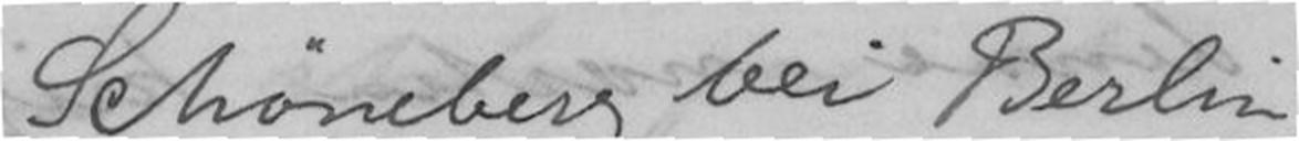Schöneberg bei Berlin
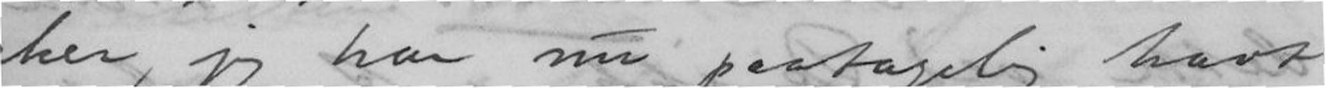sker, jeg har nu paatagelig havt
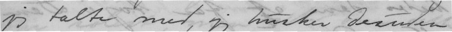jeg talte med, jeg husker desuden
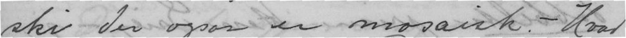ski der ogsaa er mosaisk. - Hvad
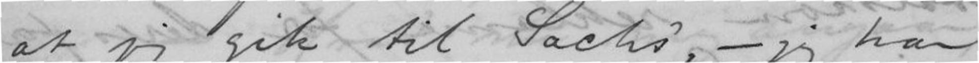at jeg gik til Sachs', - jeg har
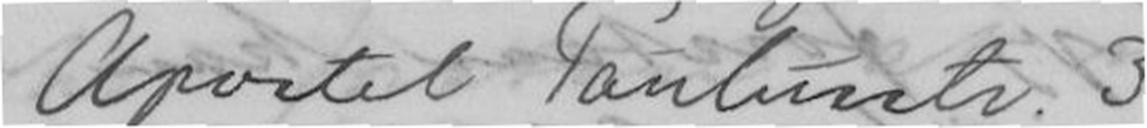Apostel Paulusstrasse 3
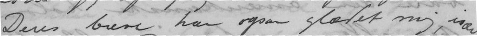Deres breve har ogsaa glædet mig, især
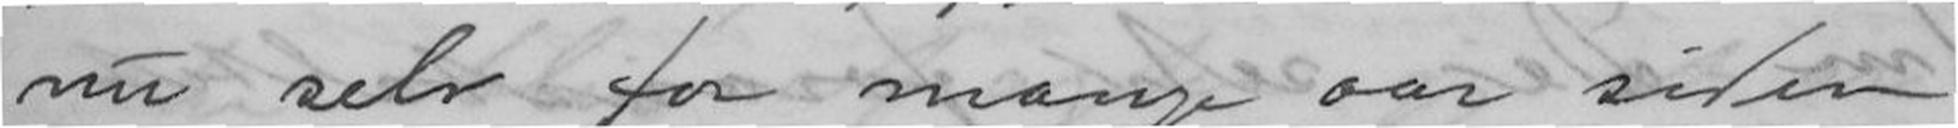nu selv for mange aar siden
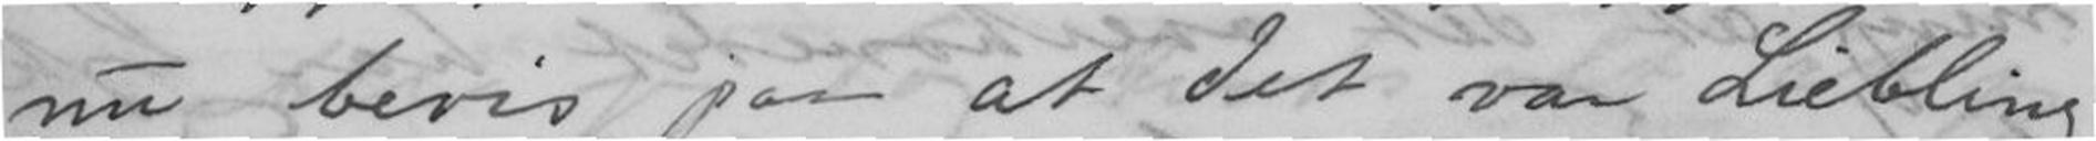nu bevis paa at det var Liebling
